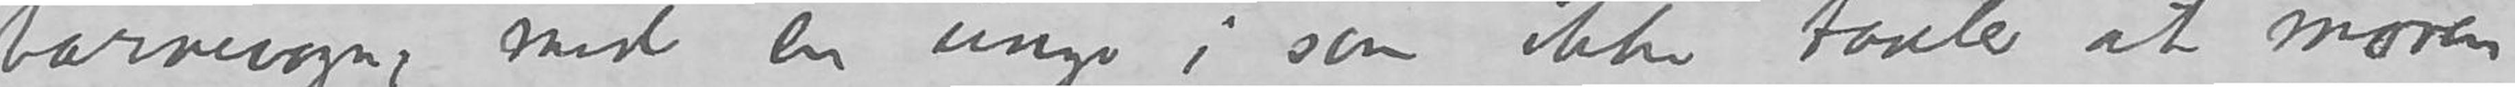barnevogn, med en unge i som ikke taaler at moren
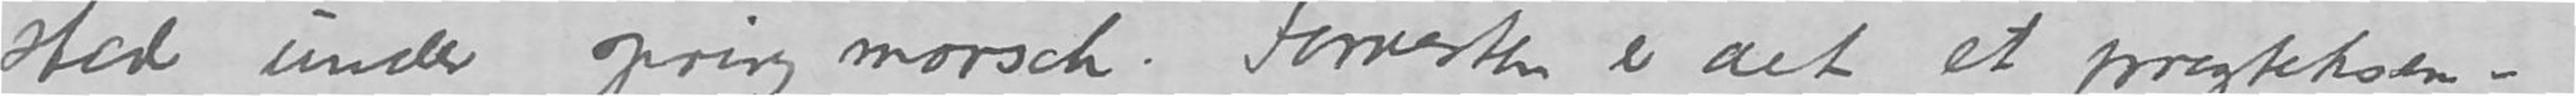sted under springmarsch. Forresten er det et prakteks-
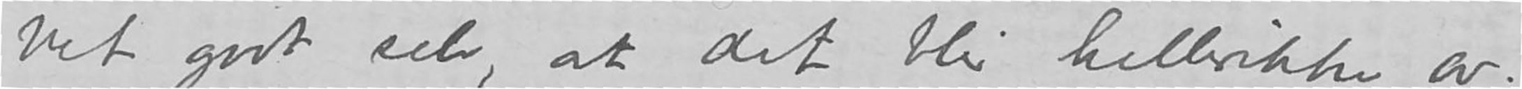vet godt selv, at det blir hellerikke av.
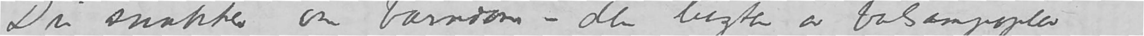Du snakker om barndom – den lugten av balsampopler
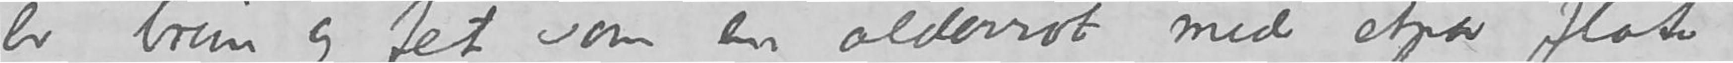er brun og fet som en olderrot med etpar flate
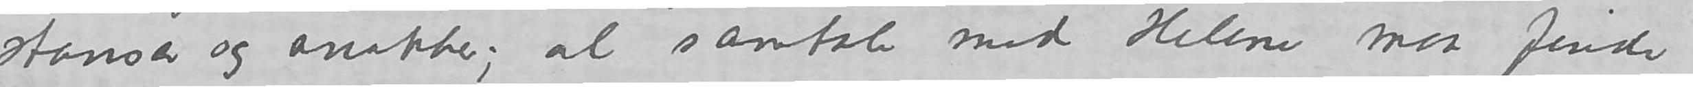stanser og snakker; al samtale med Helene maa finde
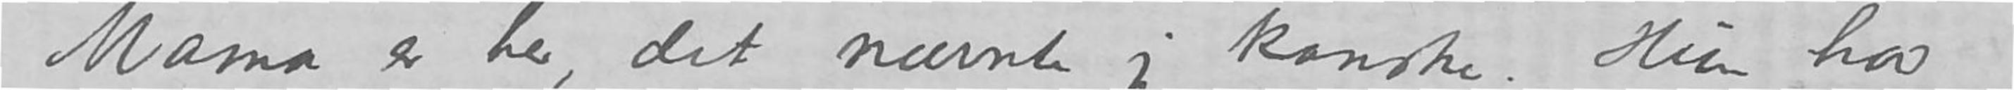Mama er her, det nævnte jeg kanske. Hun har
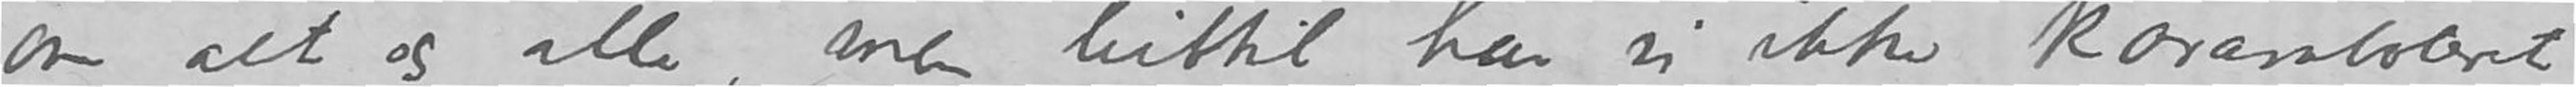om alt og alle, men hittil har vi ikke koremloteret –-
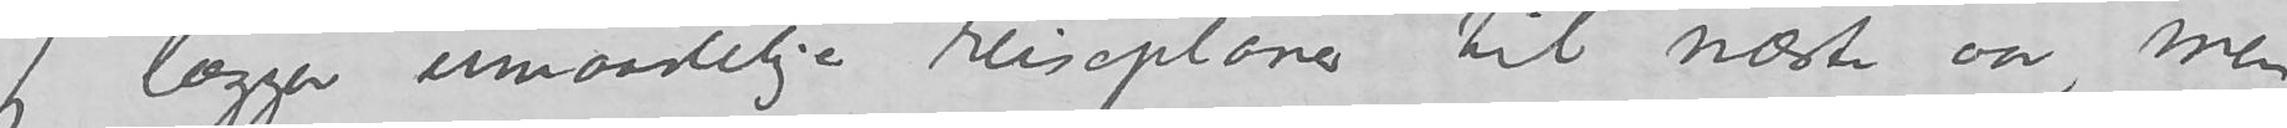Jeg lægger umaadelige reiseplaner til næste aar, men
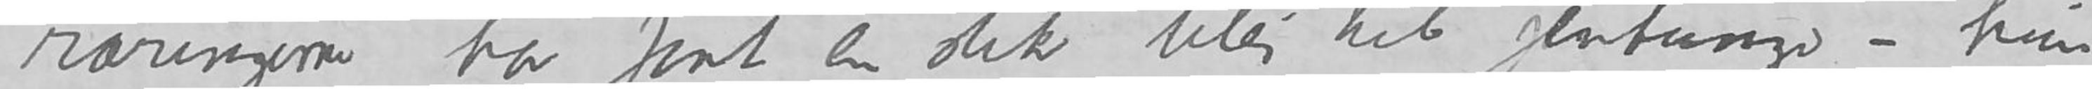raringerne har faat en slik blei til jentunge – hun
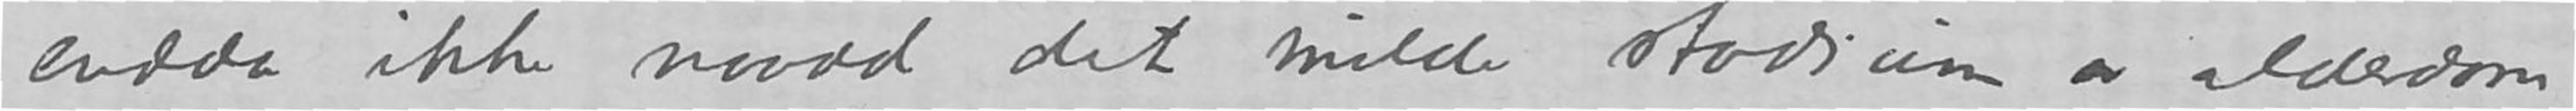endda ikke naadd det milde stadium av alderdom.
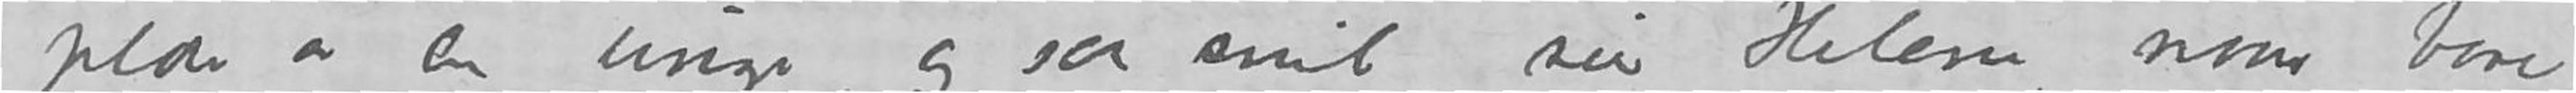emplar av en unge, og saa snil, sier Helene, naar bare
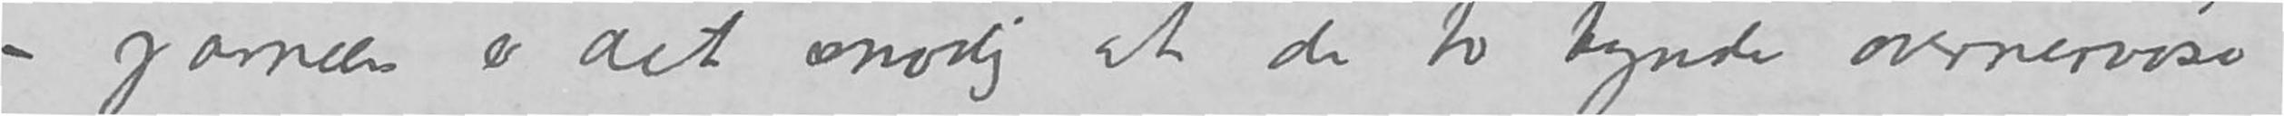jamen er det snodig at de to tynde overnervøse
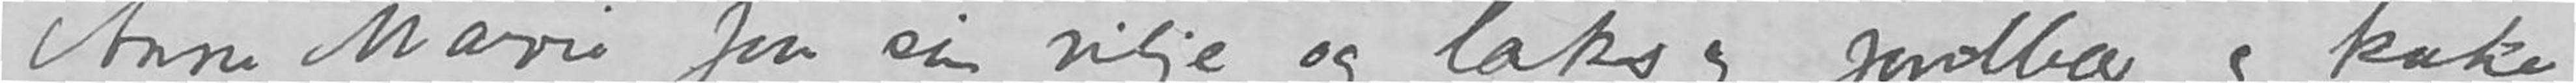Anne Marie faar sin vilje og laks og jordbær og kaker
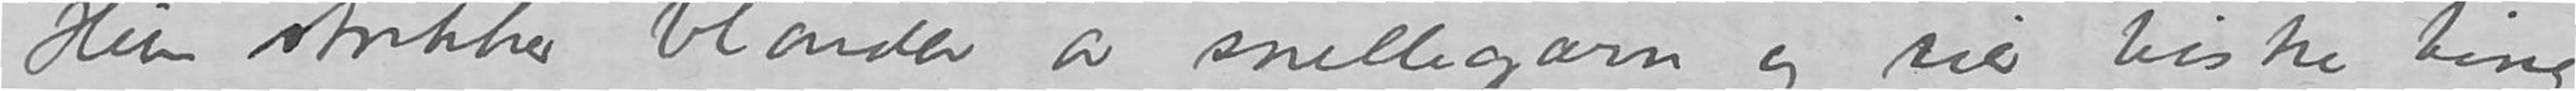Hun strikker blonder av snellegarn og sier bistre ting
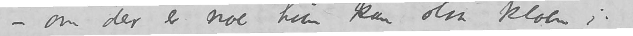- om der er noe hun kan slaa kloen i.
